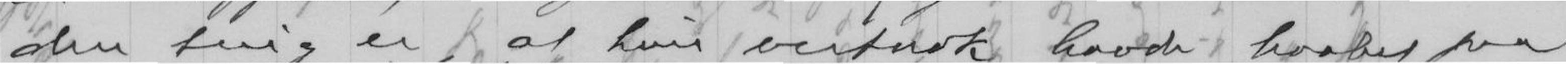den ting er, at hun vistnok havde haabet paa
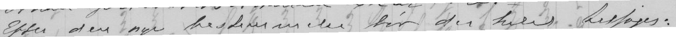Efter den nye bestemmelse bør der helst tilføjes:
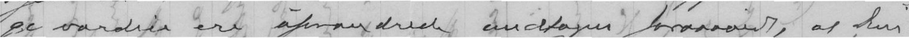ge værdier ere uforandrede undtagen forsaavidt, al Din
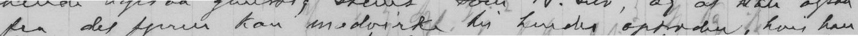fra det fjerne kan medvirke til hendes optræden, hvis han
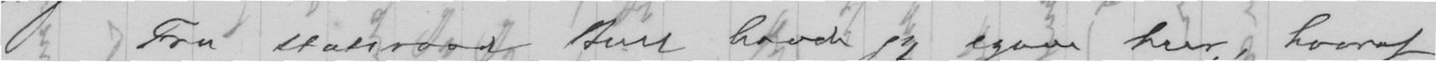Fra statsraad Bull havde jeg igaar brev, hvoraf
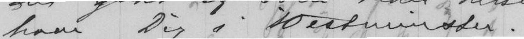have Dig i Westminster.
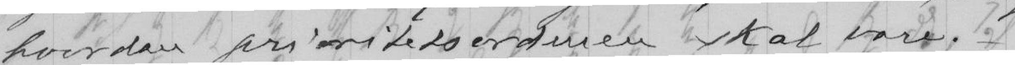hvordan prioritetsordenen skal være.
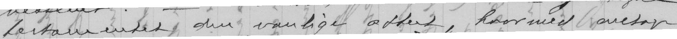testamentet, den vanlige attest, hvor med antage-
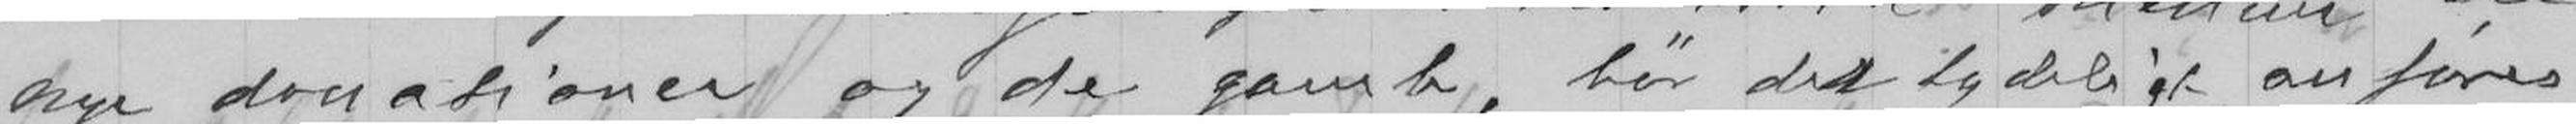nye donationer og de gamle, tør det tydeligt anføres
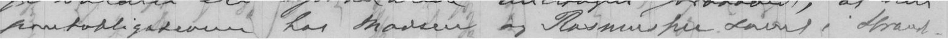pantobligationer hos Madsen og Rasmussen Lovet i Strand-
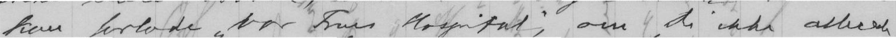kan forlade Vor Frues Hospital, om Du ikke allerede
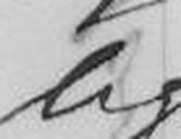lig.
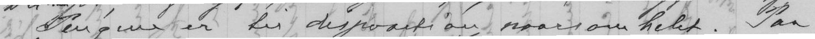Pengene er til disposition naarsom helst. Paa
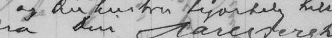fra Din Karl Bergh
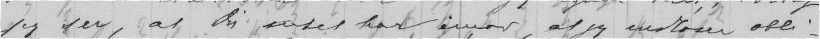jeg ser, at Du intet har imod at jeg indløser obli-
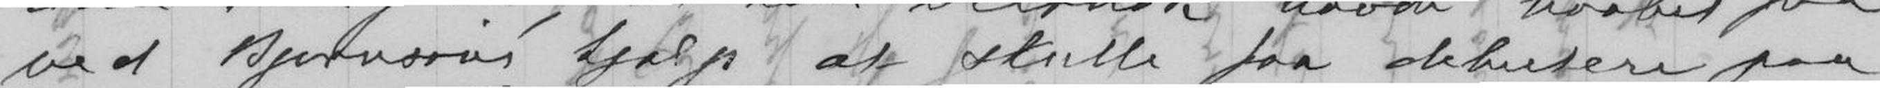ved Bjørnsons hjælp at skulle faa debutere paa
